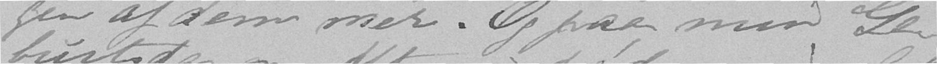gen af dem mer. Og paa min Ge-
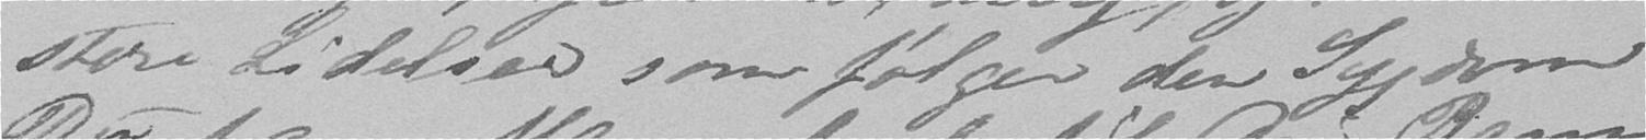store Lidelser som følger den Sygdom
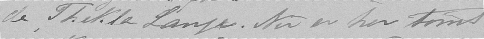de Thekla Lange. Nu er her tomt
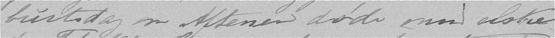burtsdag om Aftenen døde min elske-
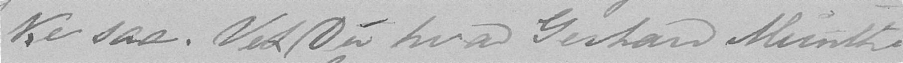ke saa. Vet Du hvad Gerhard Munthe
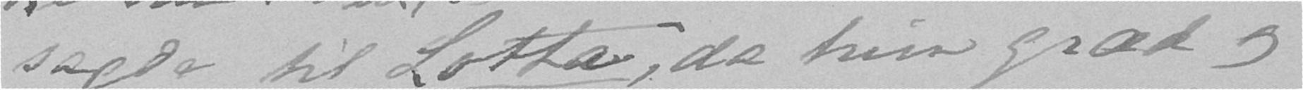sagde til Lotta, da hun græd og
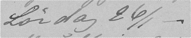Lørdag 26/1 -
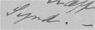Synd. -
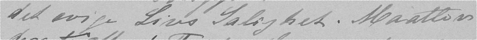det evige Livs Salighet. Maatte vi
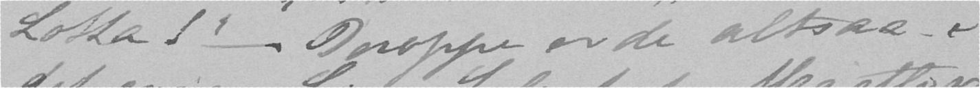Lotta!" - Deroppe er de altsaa - i
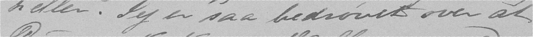heller. Jeg er saa bedrøvet over at
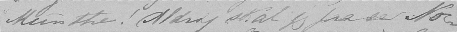Munthe! Aldrig skal jeg faa se No-
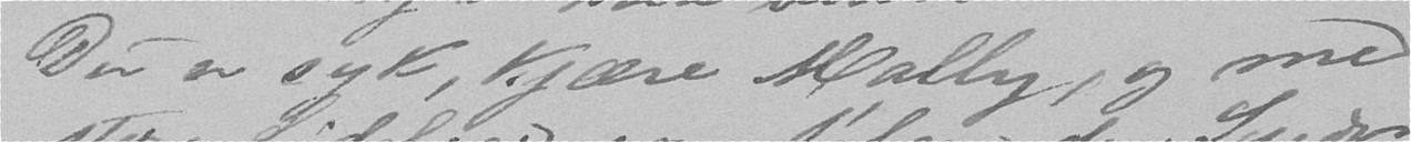Du er syk, kjære Mally, og med
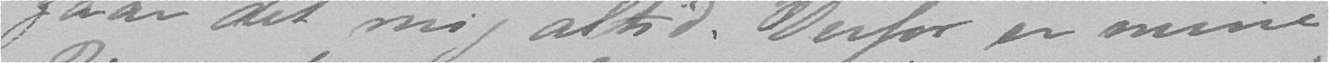gaar det mig altid. Derfor er mine
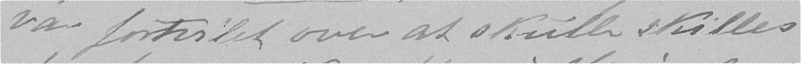var fortvilet over at skulle skilles
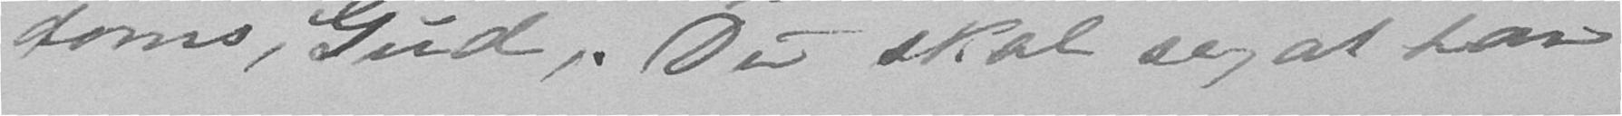doms, Gud,. Du skal se, at han
